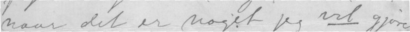naar det er noget jeg vil gjøre;
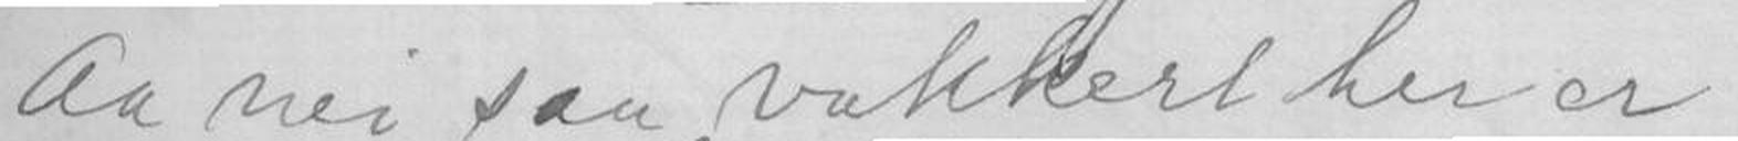Aa nei saa vakkert her er!
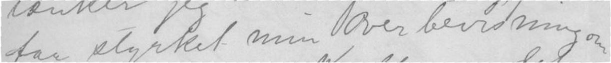faa styrket min Overbevisning om
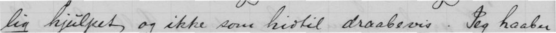lig hjulpet og ikke som hidtil draabevis. Jeg haaber
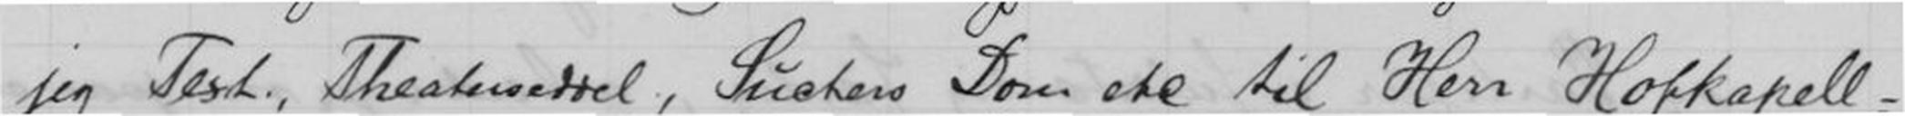jeg Text, Theaterseddel, Sucher Dom etc til Herr Hofkapell-
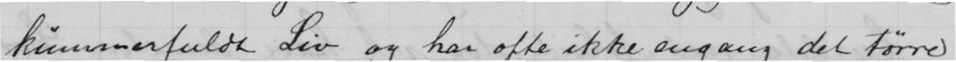kummerfuldt Liv og har ofte ikke engang det tørre
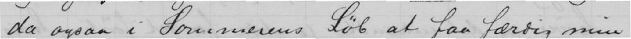da ogsaa i Sommerens Løb at faa færdig min
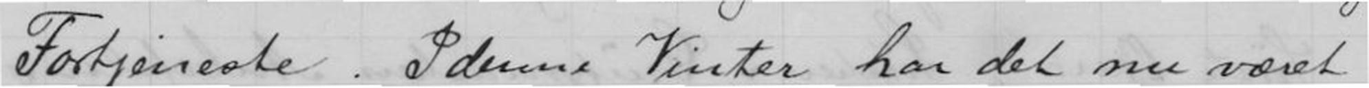Fortjeneste. I denne Vinter har det nu været
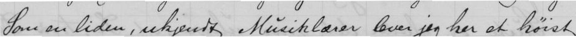Som en liden, ukjendt Musiklærer lever jeg her et høist
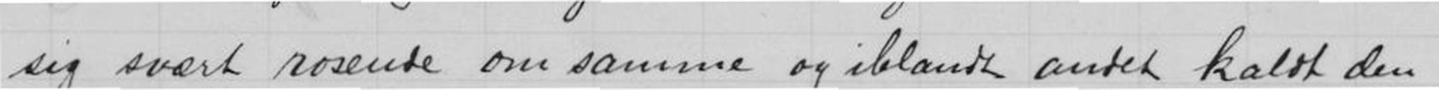sig svært rosende om samme og iblandt andet kaldt den
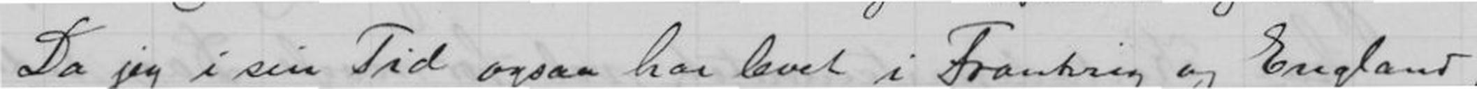Da jeg i sin Tid ogsaa har levet i Frankrig og England,
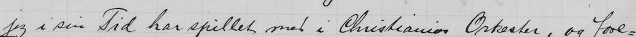jeg i sin Tid har spillet med Christiania Orkester, og fore-
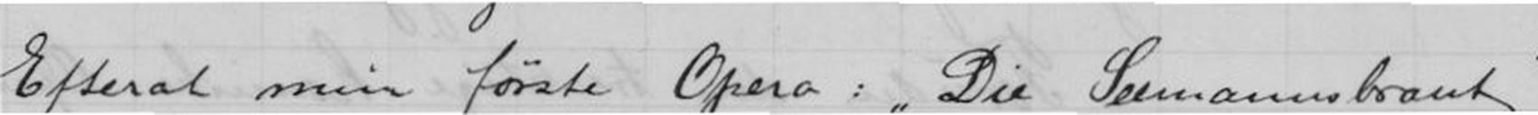Efterat min første Opera: Die Seemannsbraut
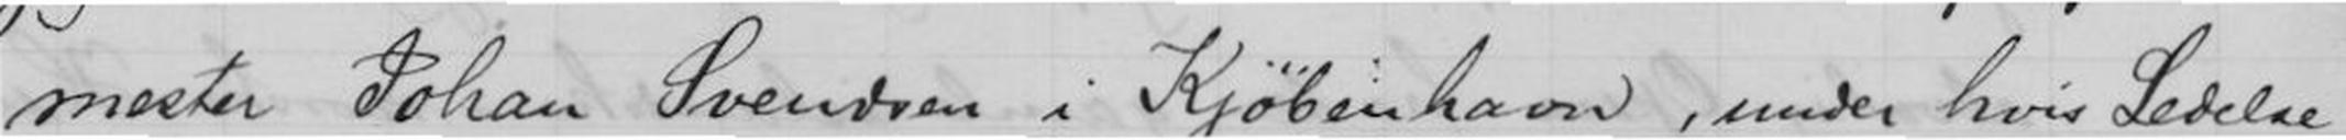mester Johan Svendsen i Kjøbenhavn, under hvis Ledelse
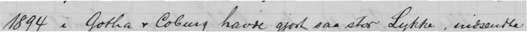1894 i Gotha & Coburg havde gjort saa stor Lykke indsendte
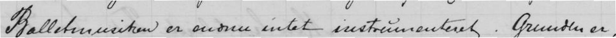Balletmusiken er endnu intet instrumenteret. Grunden er,
